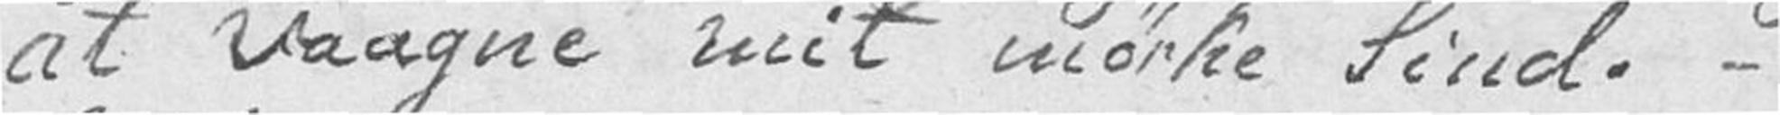at vaagne mit mørke Sind. –
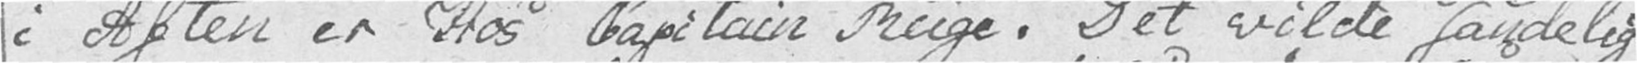i Aften er Hos Capitain Ruge. Det vilde sandelig
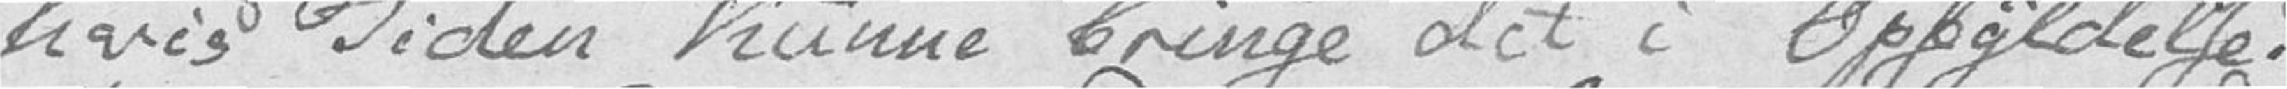hvis Tiden kunne bringe det i Opfyldelse.
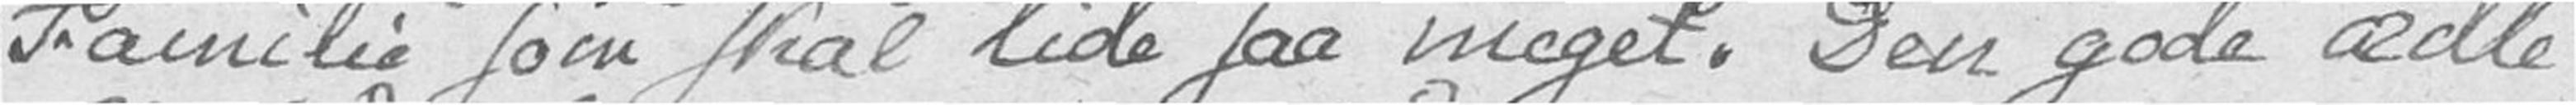Familie som skal lide saa meget. Den gode ædle
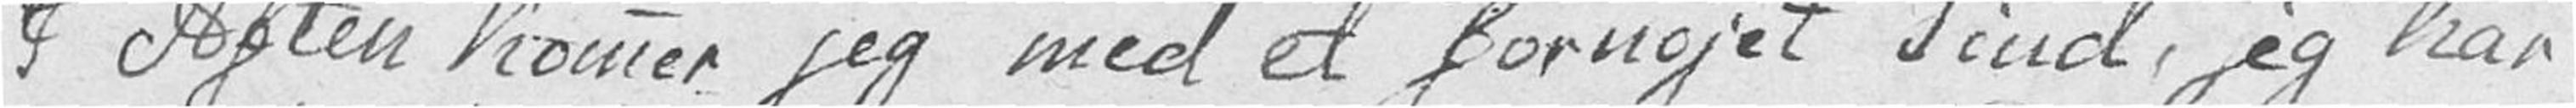I Aften komer jeg med et fornøjet Sind, jeg har
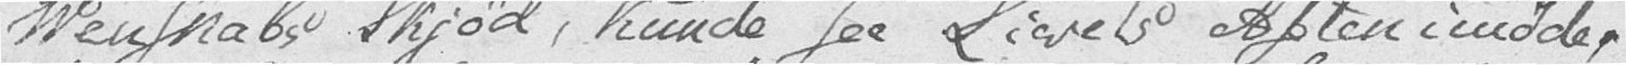Venskabs Skjød, kunde see Livets Aften imøde.
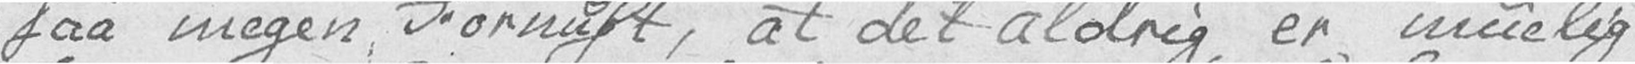saa megen Fornuft, at det aldrig er muelig
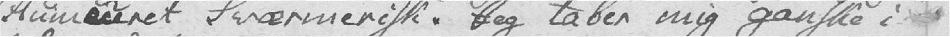Humeuret Sværmerisk. Jeg taber mig ganske i
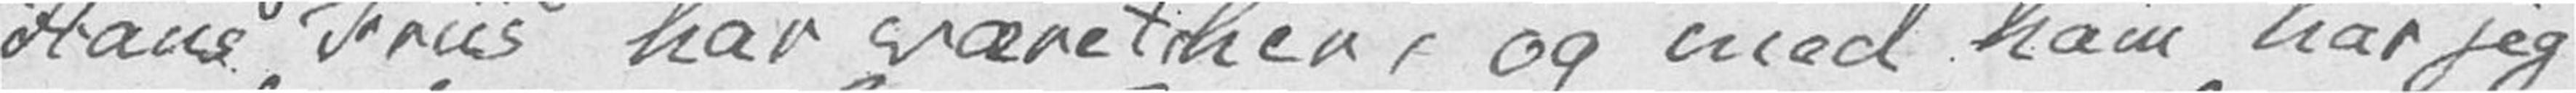Hans Friis har været her, og med ham har jeg
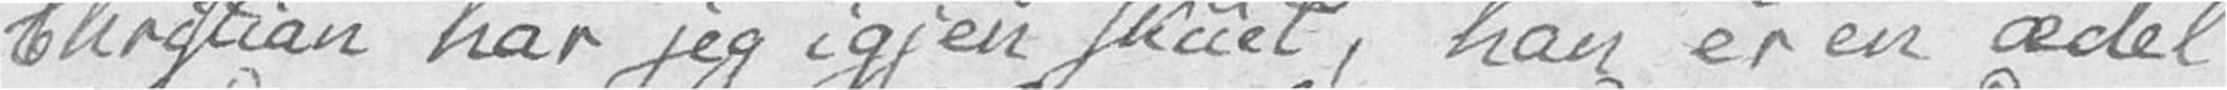Christian har jeg igjen skuet, han er en ædel
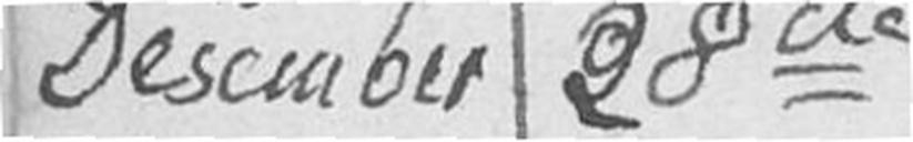Desember 28de
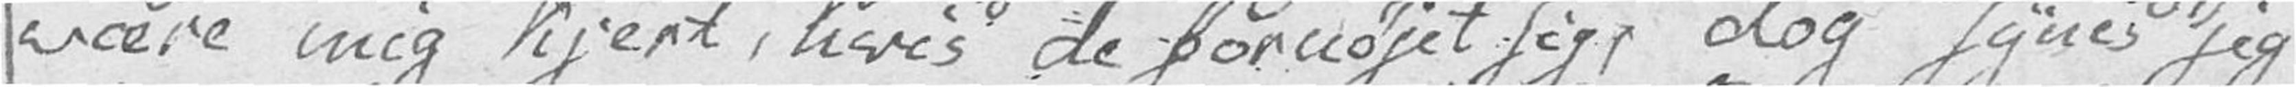være mig kjert, hvis de fornøjet sig, dog synes jeg
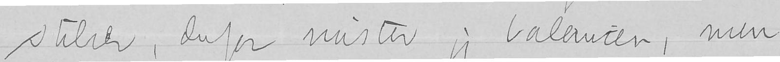stelser, derfor mister jeg balancen, men
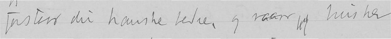forstaar du kanske bedre, og naar jeg husker
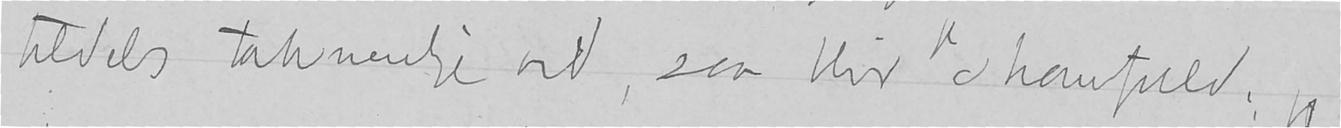tildels taknemlige ord, saa blir jeg skamfuld, jeg
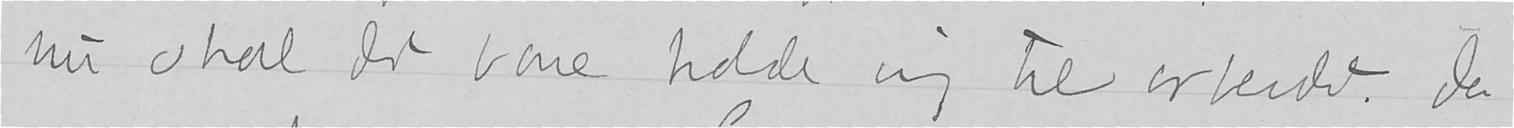nu skal det bare holde mig til arbeidet. Ja
